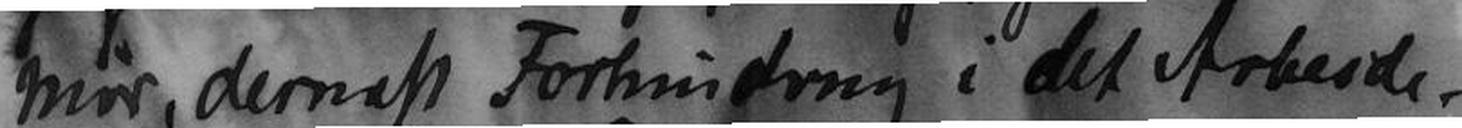mør, dernest Forhindring i det Arbeide -
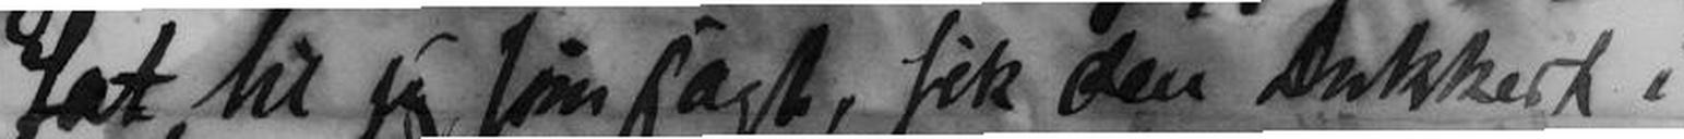sat, til jeg som sagt, fik den Dukkert i
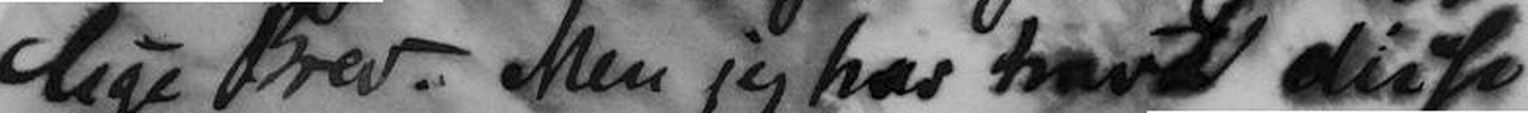lige Brev. Men jeg har havt disse
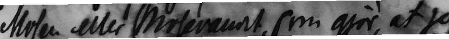Mosen eller Mosevandet, som gjør at jeg
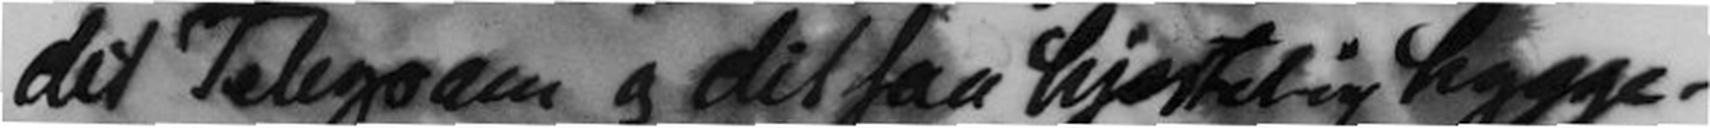dit Telegram og dit saa hjærtelig hygge-
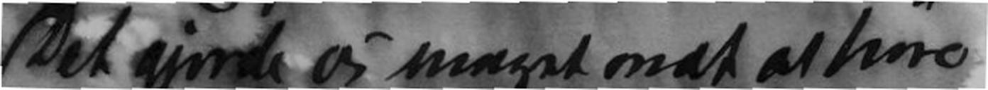Det gjorde os meget mat at høre
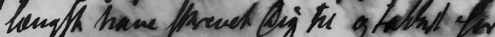længst have skrevet Dig til og takket for
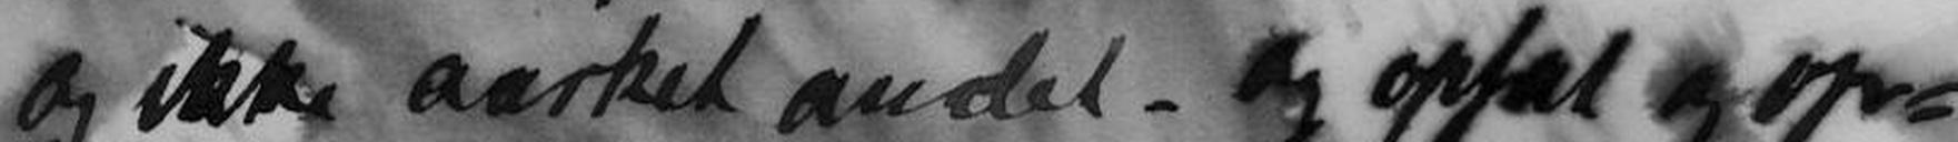og ikke aarket andet. Og opsat og op-
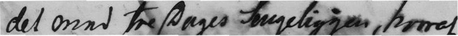det med tre Dages Sengeligge, hvoraf
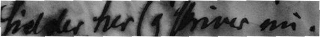sidder her og skriver nu.
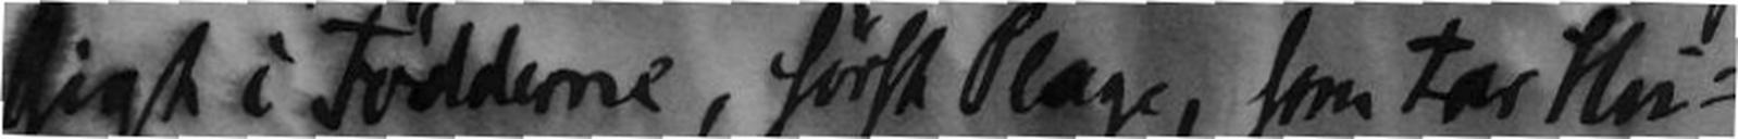Gigt i Fødderne, først Plage, som tar Hu-
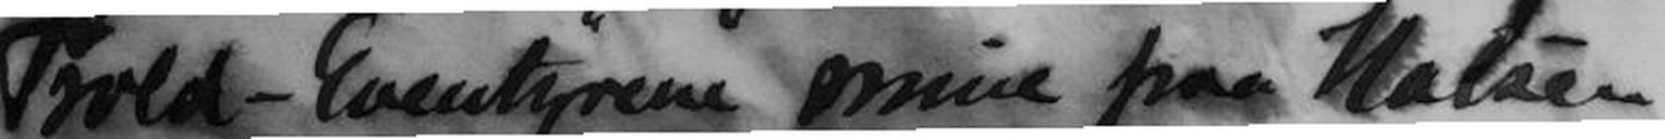Trold-Eventyrene mine paa Halsen
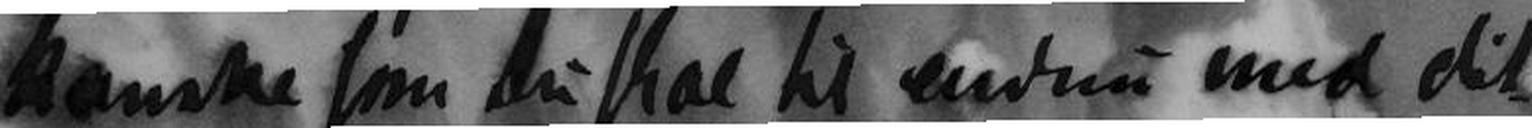kanske som Du skal til endnu med dit
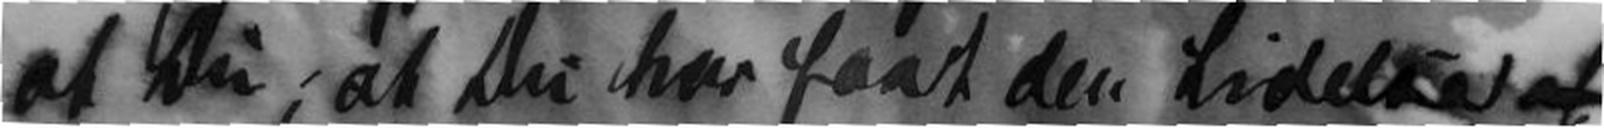at Du, at Du har faat den lidelse af
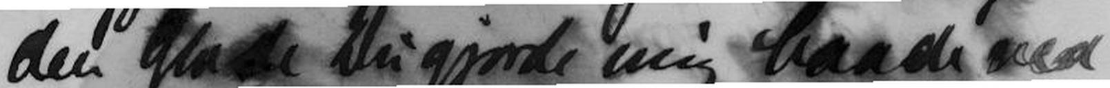den Glæde Du gjorde mig baade ved
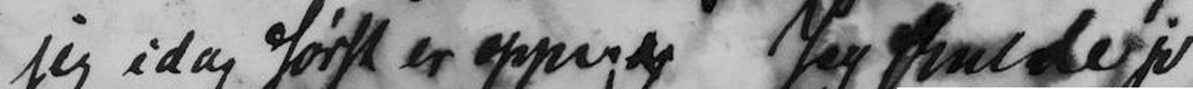jeg idag først er oppe og Jeg skulde jo
